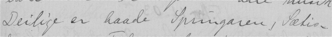Deilige er haade Springaren, Sætis-
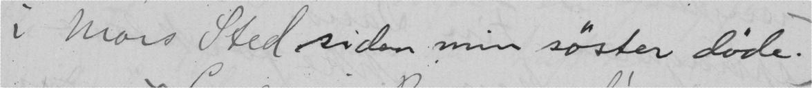i mors Sted siden min søster døde.
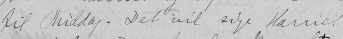til Middag. Det vil sige Harriet
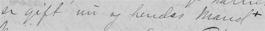er gift nu og hendes Mandx
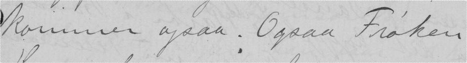kommer ogsaa. Ogsaa Frøken
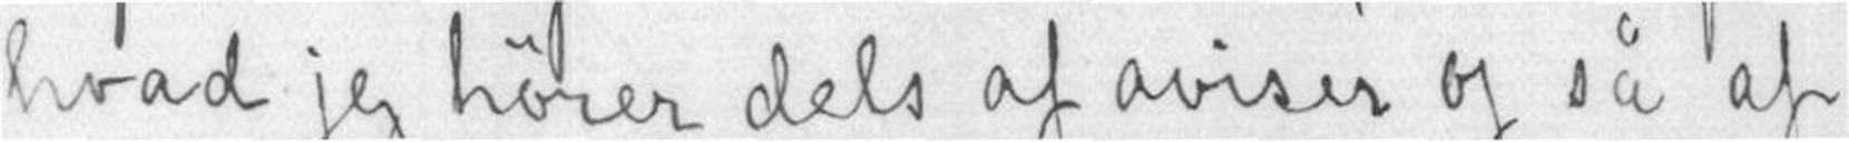hvad jeg hører dels af aviser og så af
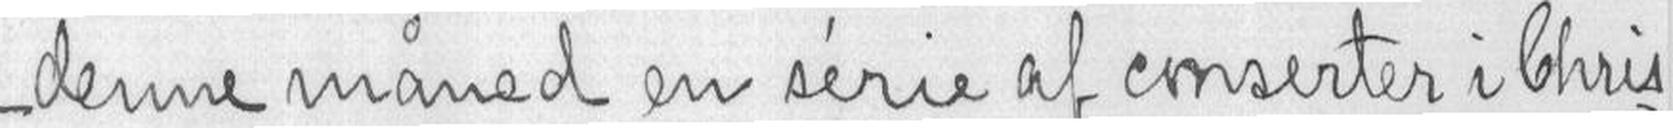-denne måned en série af conserter i Chris-
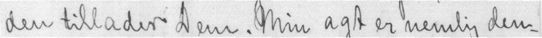den tillader Dem. Min agt er nemlig den -
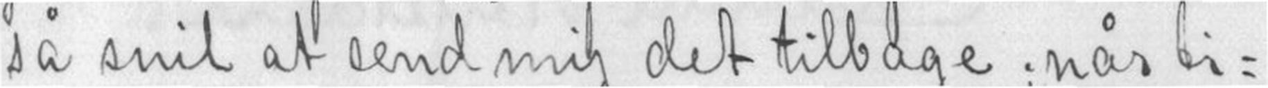så snil at send mig det tilbage; når ti-
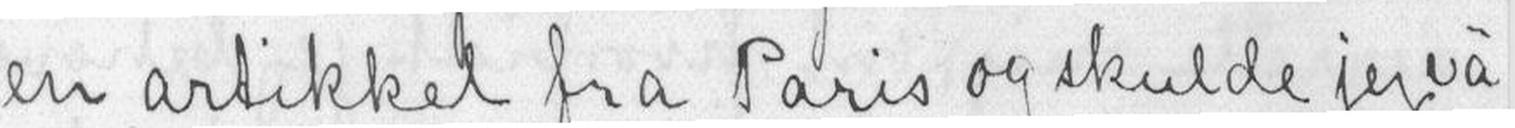en artikkel fra Paris og skulde jeg vä-
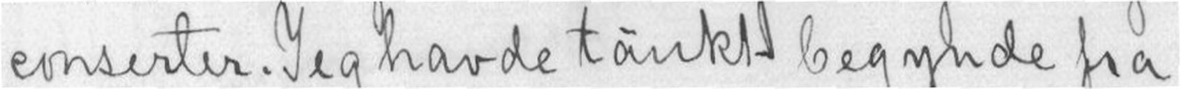conserter. Jeg havde tänkt begynde fra
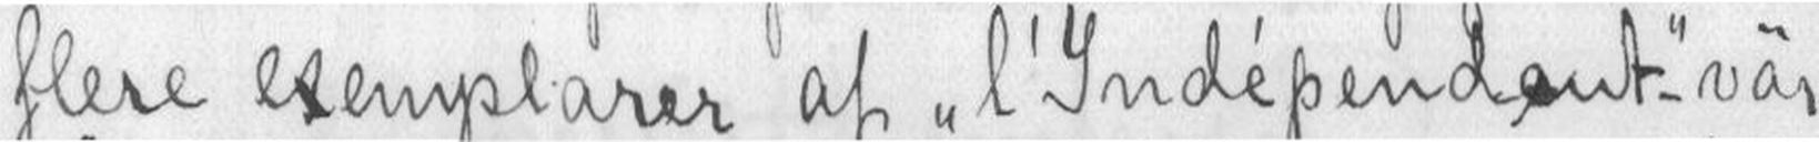flere exemplarer af l'Indépendent, vär
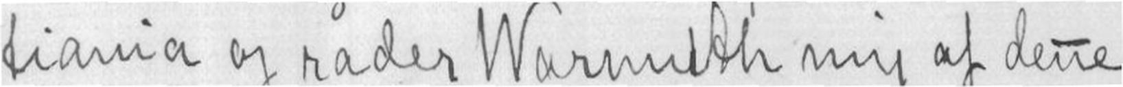tiania og råder Warmuth mig af denne
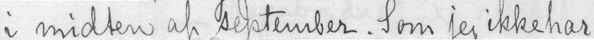i midten af september. Som jeg ikke har
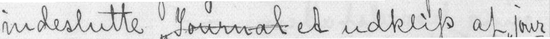indeslutte "Journal et udklip af "jour-
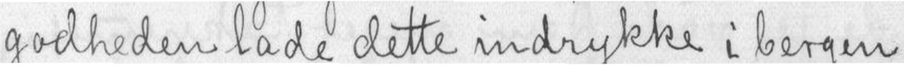godheden lade dette indrykke i bergen-
